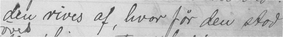den rives af, hvor før den stod
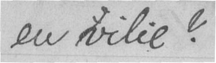en vilie?
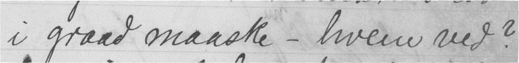i graad maaske - hvem ved?
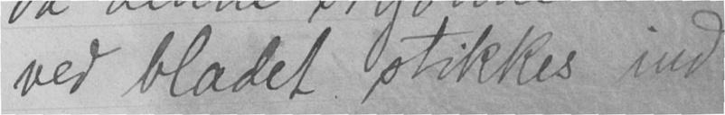ved bladet stikkes ind
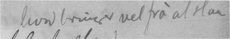hvad bringer vel fra al rlaa
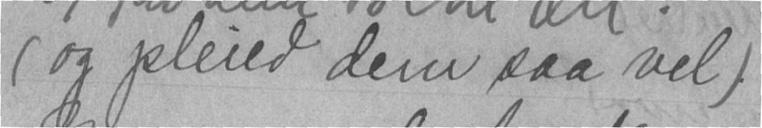(og pleied dem saa vel)
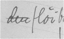den fløi bort,
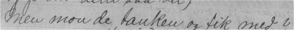Men mon de tanken og fik med?
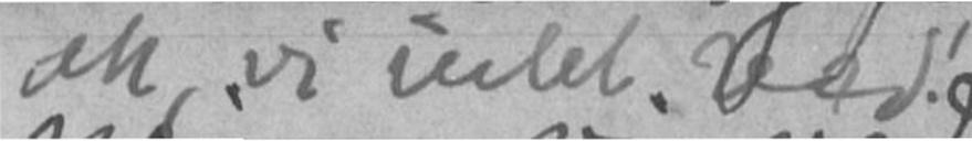ak, vi intet ved!
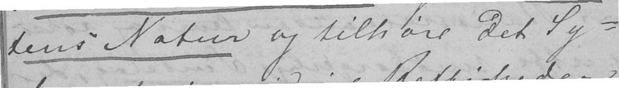tens Natur og tilhøre det Sy-
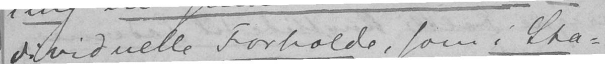dividuelle Forholde, som i Sta-
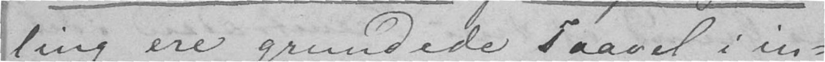ling ere grundede saavel i in-
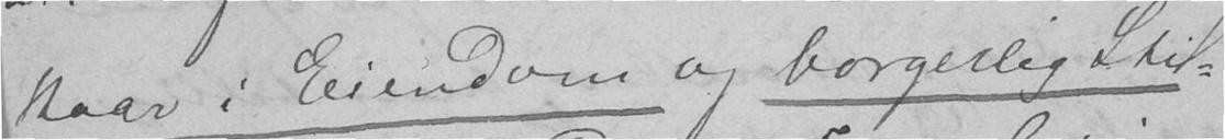kaar i Eiendom og borgerlig Stil-
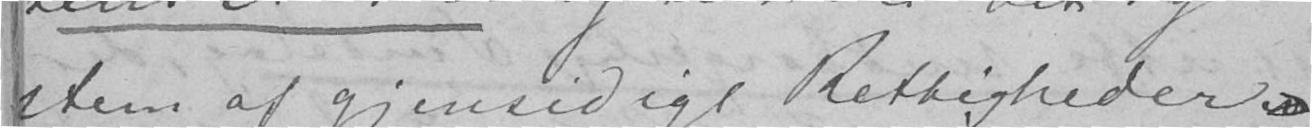stem af gjensidige Rettigheder,
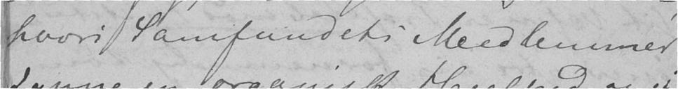hvori Samfundets Medlemmer
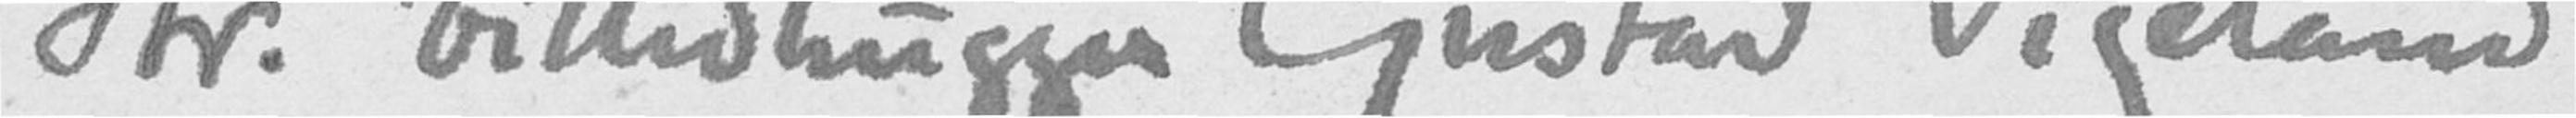Hr. billedhugger Gustav Vigeland
